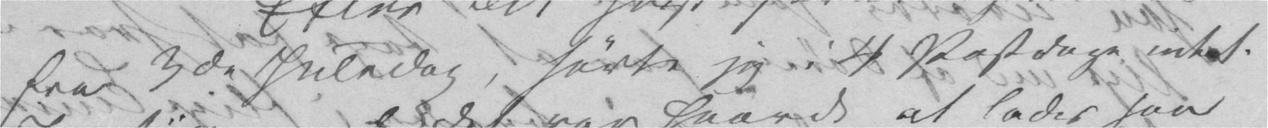fra 3de Juledag, hørte jeg i 4 Postdage intet.
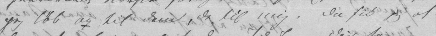jeg løb op til dem, de til mig, da fik jeg et
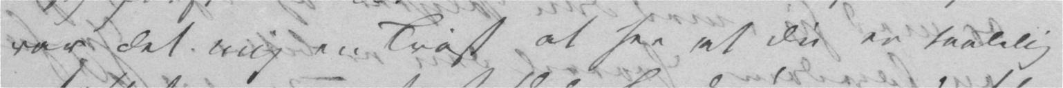var det mig en Trøst at see at Du er taalelig
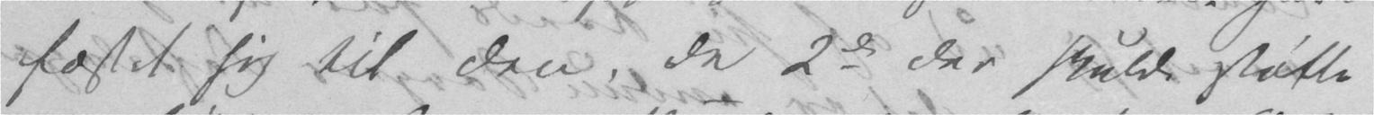fæstet sig til den, de 2de der skulde støtte
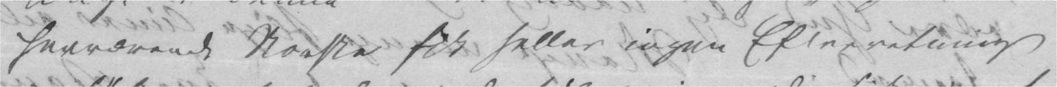herværende Norske fik heller ingen Efterretning
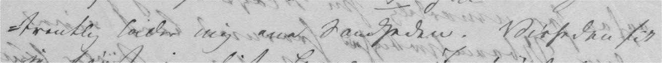trentlig lader mig ane Sandheden. Visheden fik
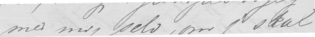med mig selv, om jeg skal
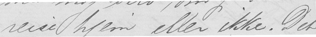reise hjem eller ikke. Det
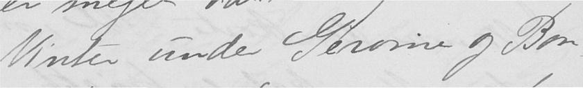Vinter under Gerome og Bon-
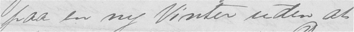paa en ny Vinter uden at
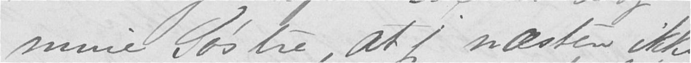mine Søstre, at jeg næsten ikke
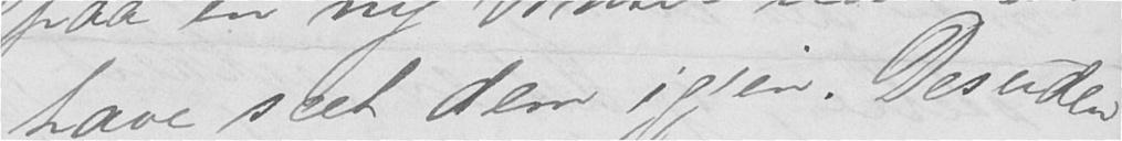have seet dem igjen. Desuden
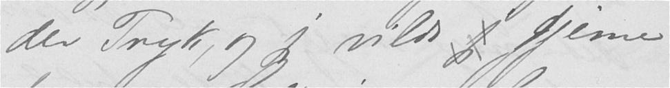der Tryk, og jeg vilde jeg gjerne
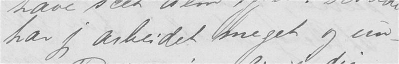har jeg arbeidet meget og un-
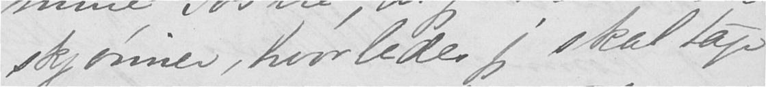skjønner, hvorledes jeg skal tage
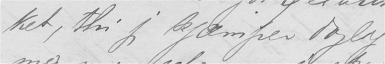ket, thi jeg kjæmper daglig
